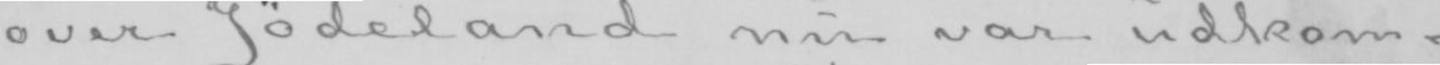over Jødeland nu var udkom-
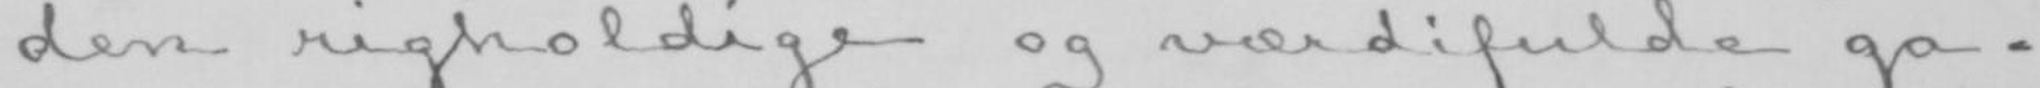den righoldige og værdifulde ga-
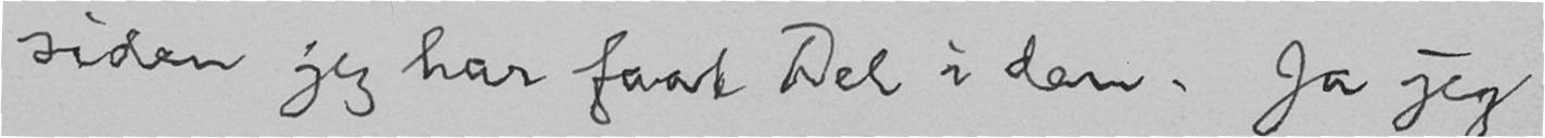siden jeg har faat Del i den. Ja jeg
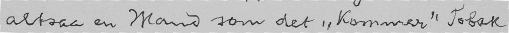altsaa en Mand som det "kommer" Tobak
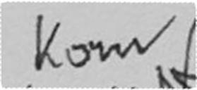kom
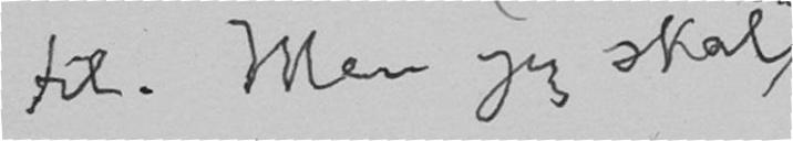til. Men jeg skal
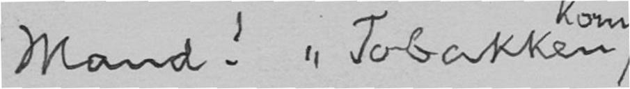Mand! "Tobakken
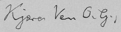Kjære Ven O. G.,
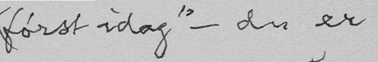først idag" - du er
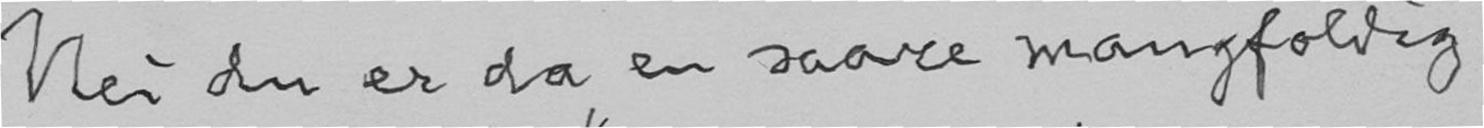Nei du er da en saare mangfoldig
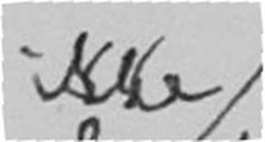ikke
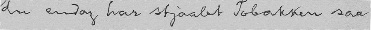du endog har stjaalet Tobakken saa
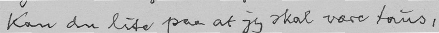kan du lite paa at jeg skal være taus,
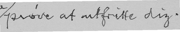prøve at utfritte dig.
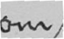om
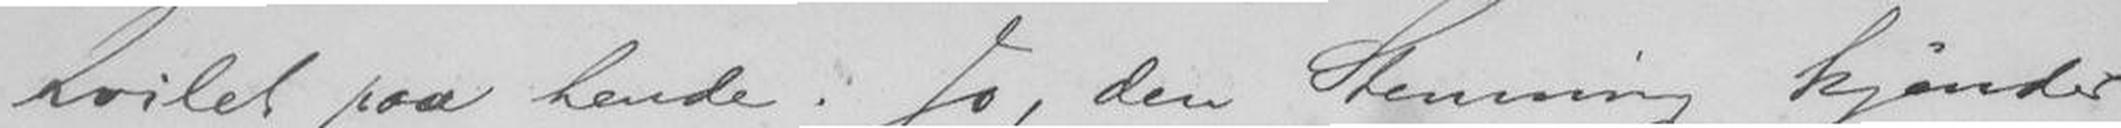hvilet paa hende. Jo, den Stemning kjender
